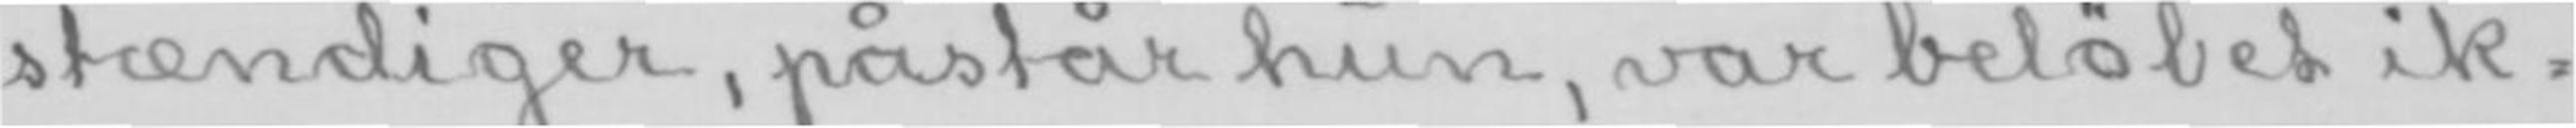stændiger, påstår hun, var beløbet ik-
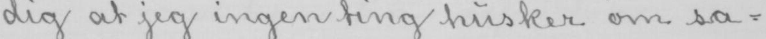dig at jeg ingen ting husker om sa-
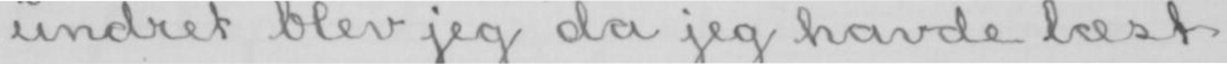undret blev jeg da jeg havde læst
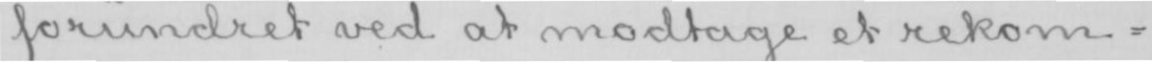forundret ved at modtage et rekom-
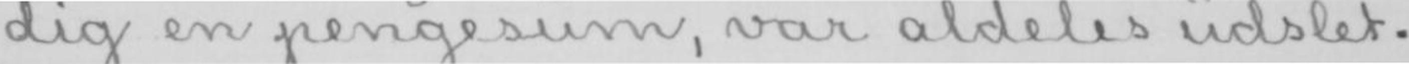dig en pengesum, var aldeles udslet-
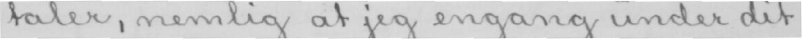taler, nemlig at jeg engang under dit
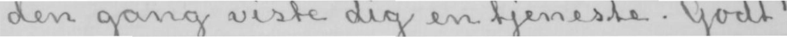den gang viste dig en tjeneste. Godt!
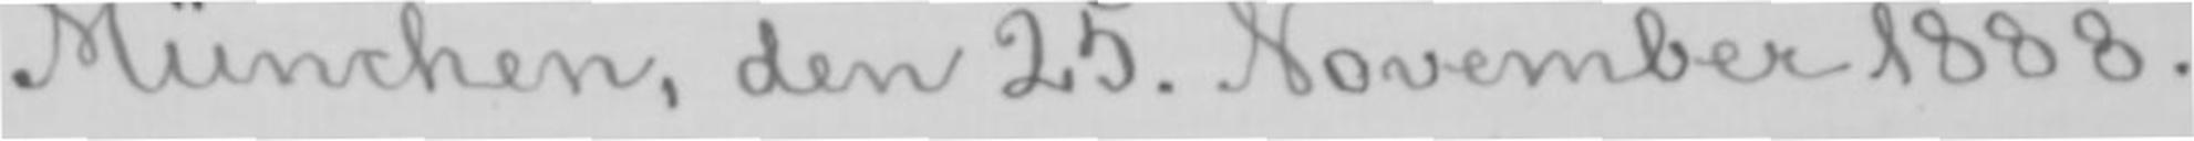München, den 25. November 1888.
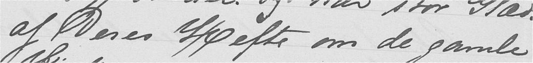af Deres Hefte om de gamle
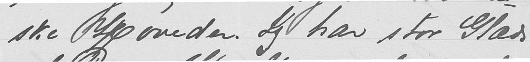ske Hoveder. Jeg har stor Glæde
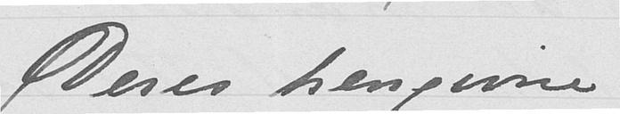Deres hengivne
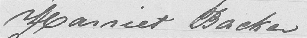Harriet Backer
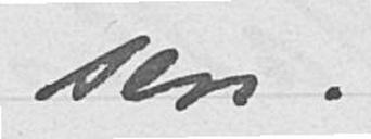sen.
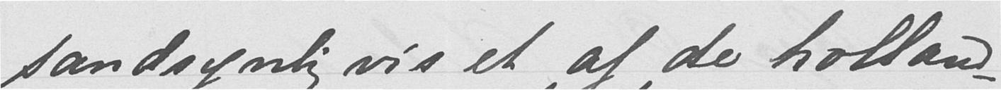sandsynligvis et af de holland-
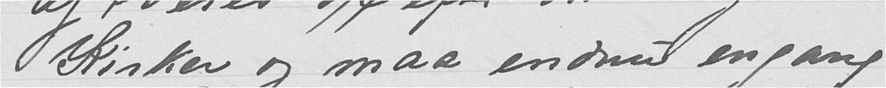Kirker og maa endnu engang
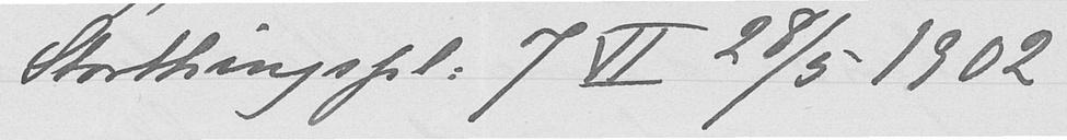Storthingspl. 7 VI 28/5 1902
